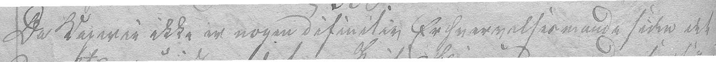Da Kaperier ikke er nogen definitiv Erhvervelsesmaade siden det
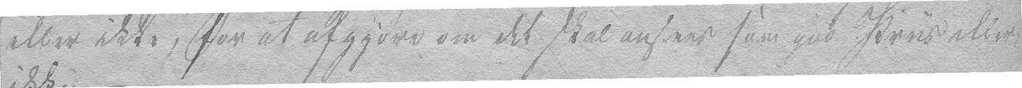eller ikke, for at afgjøre om det skal ansees som god Priis eller
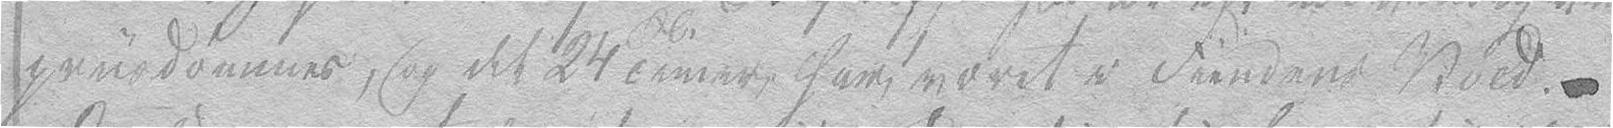priisdømmes, og det 24 Timer har været i Fiendens Vold.–
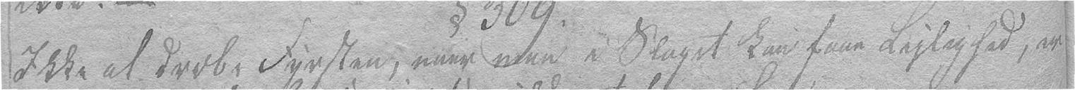Ikke at dræbe Fyrsten, naar man i Slaget kan faae Leilighed, er
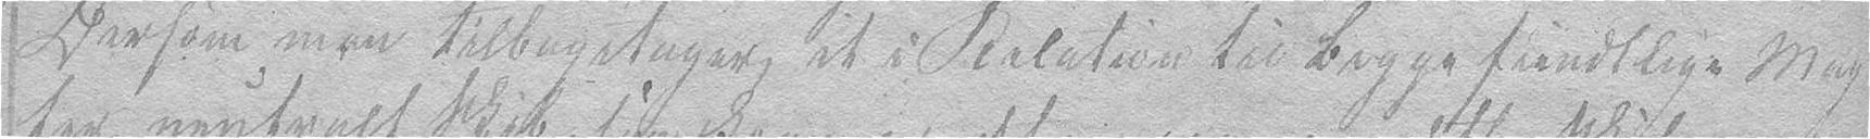Dersom man tilbagetager et i Relation til begge fiendtlige Mag-
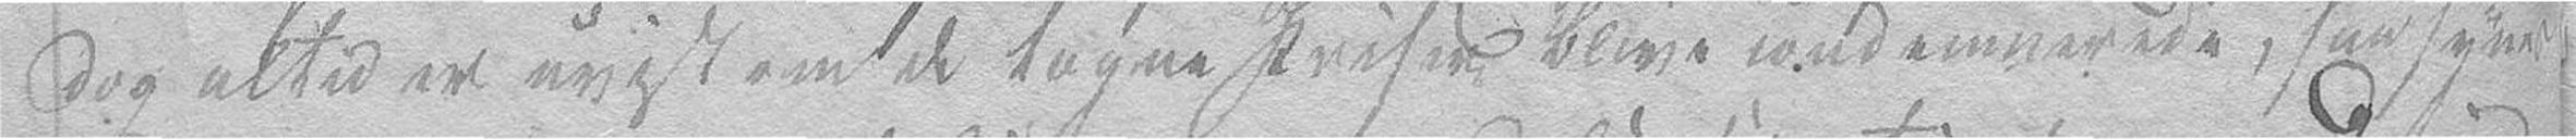dog altid er uvist om de tagne Priser blive condemnerede, saa synes
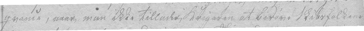qvence, naar man ikke tillader Krigeren at berøve Skibsfolkene
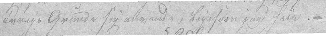øvrige Grunde sig anvende, ligesom paa hiin. –
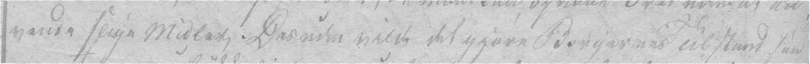vende slige Midler. Desuden vilde det gjøre Borgernes Tilstand saa
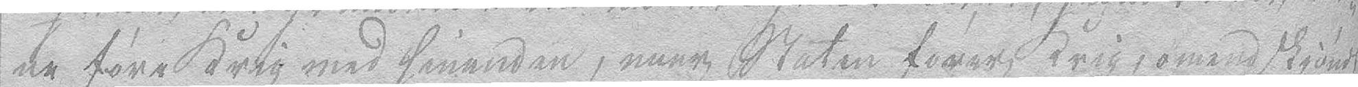ne føre Krig med hinanden, naar Staten fører Krig, omendskjøndt
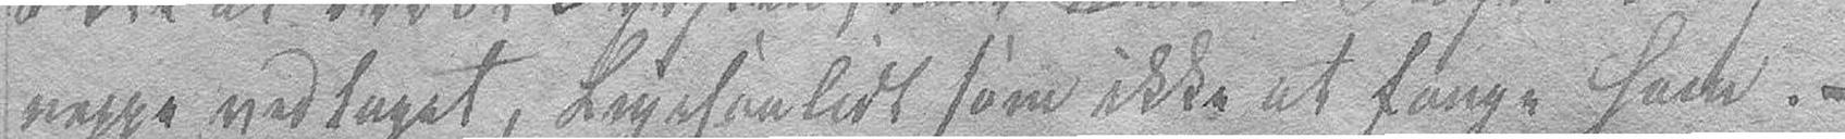neppe vedtaget, Ligesaalidt som ikke at fange ham.–
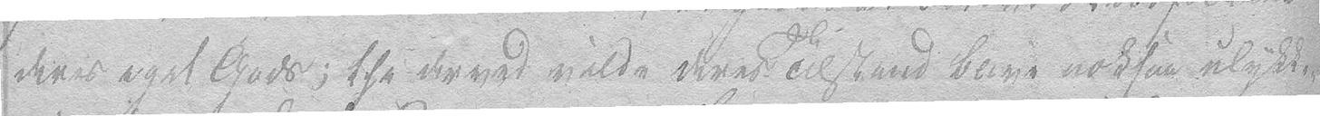deres eget Gods; thi derved vilde deres Tilstand blive noksaa ulykke-
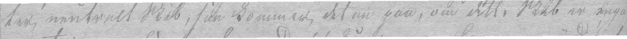ter neutralt Skib, saa kommer det an paa, om dette Skib er inge-
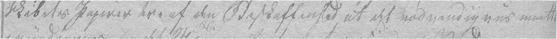Skibets Papirer ere af den Beskaffenhed at det nødvendigviis maatte
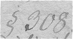§ 308.
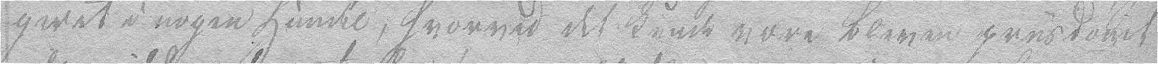geret i nogen Handel, hvorved det kunde være bleven priisX
